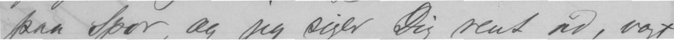paa Spor, og jeg siger Dig rent ud, vogt
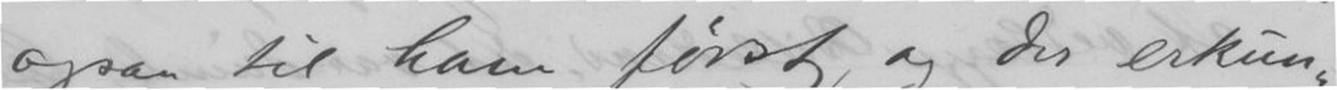ogsaa til ham først, og der erkundige
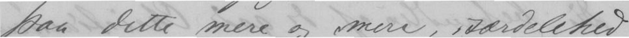paa dette mere og mere, isærdeleshed
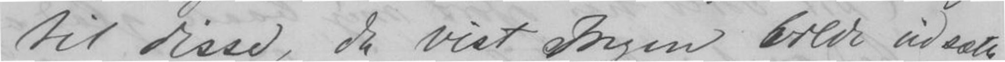til disse, da vist Ingen vilde udsatte
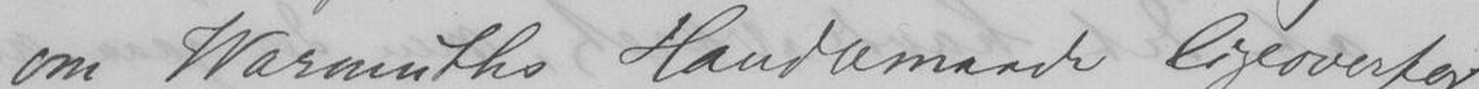om Warmuths Handlemaade ligeoverfor
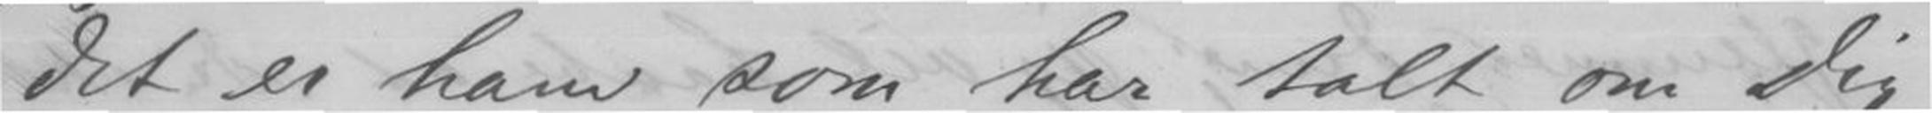Det er ham som har talt om Dig
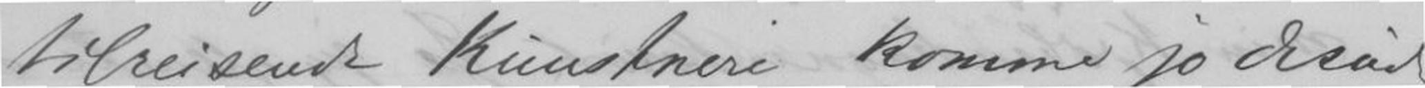tilreisende Kunstnere kommer jo desuden
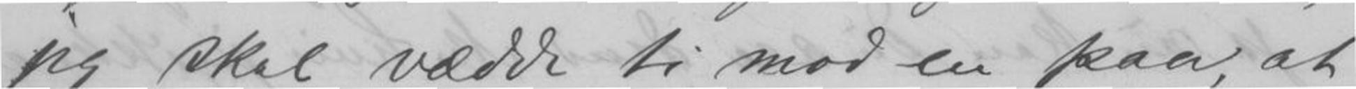jeg skal vædde ti mod en paa at
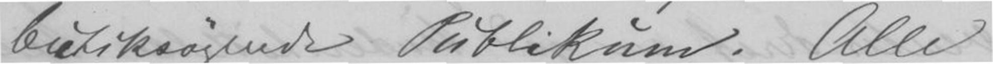butiksøgende Publikum. Alle
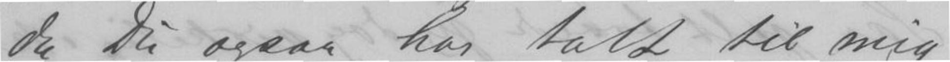da Du ogsaa har talt til mig
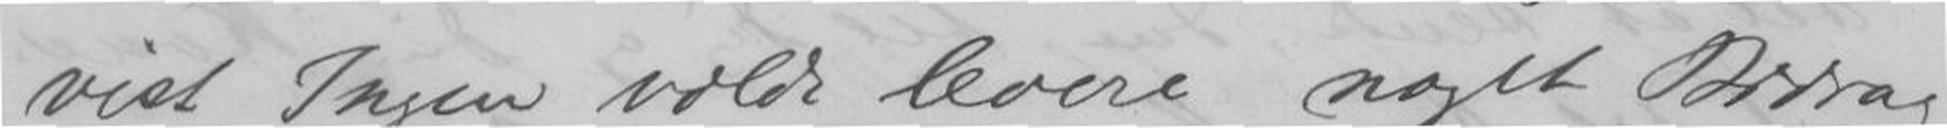vist Ingen vilde levere noget Biddrag
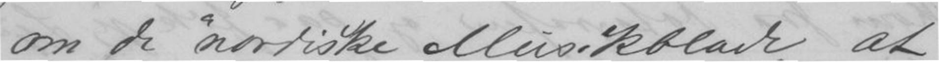om de "nordiske Musikblade" at
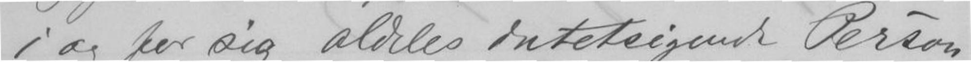i og for sig aldeles intetsigende Person
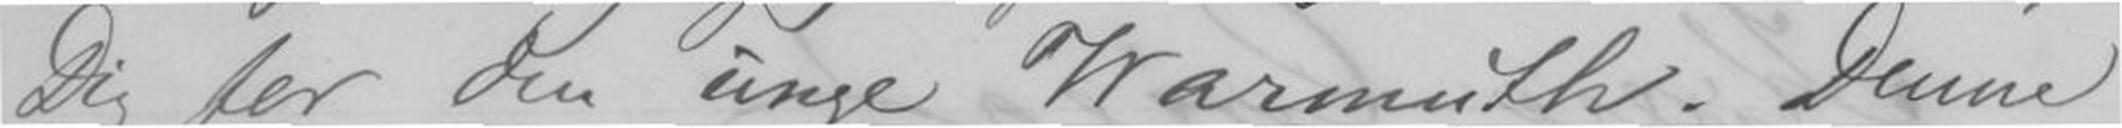Dig for den unge Warmuth. Denne
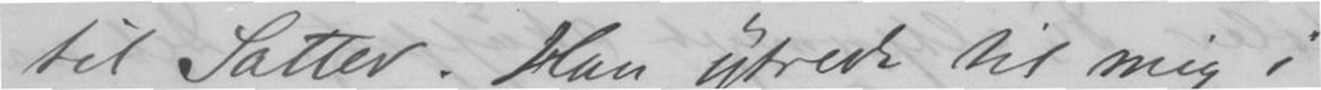til Satter. Han ytrede til mig i
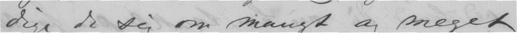de sig om mangt og meget,
